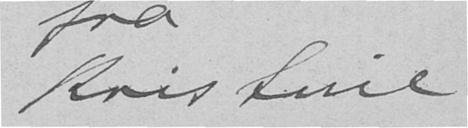Kristine
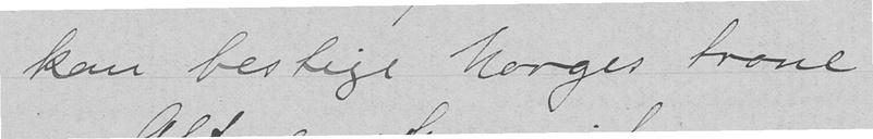kan bestige Norges trone.
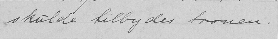skulde tilbydes tronen.
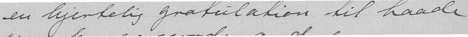en hjertelig gratulation til baade
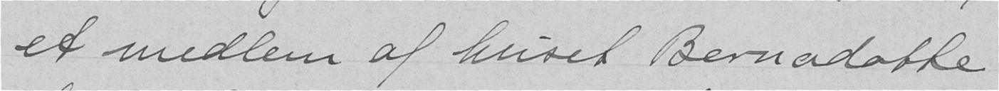et medlem af huset Bernadotte
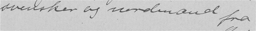svensker og nordmænd fra
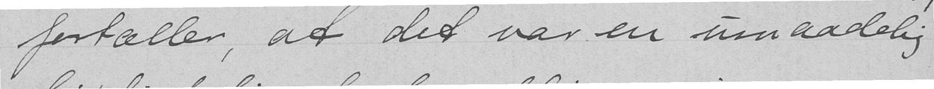fortæller, at det var en umaadelig
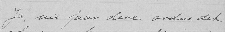Ja, nu faar dere ordne det
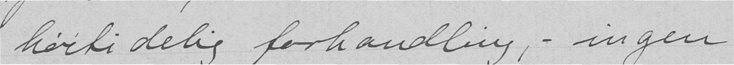høitidelig forhandling, - ingen
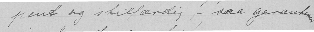pent og stilfærdig, - saa garanterer
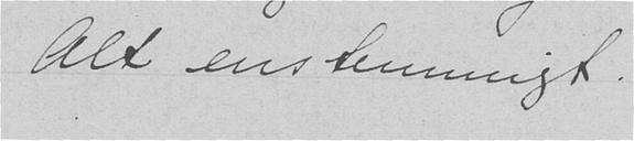Alt enstemmigt.
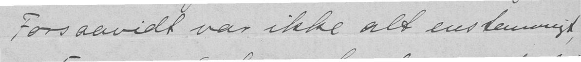Forsaavidt var ikke alt enstemmigt,
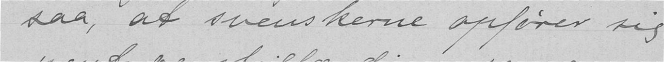saa, at svenskerne opfører sig
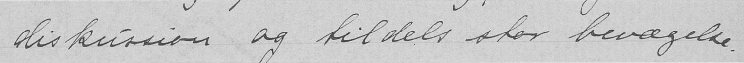diskussion og tildels stor bevægelse.
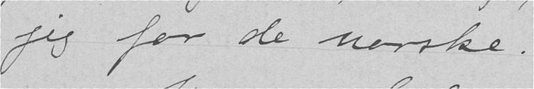jeg for de norske!
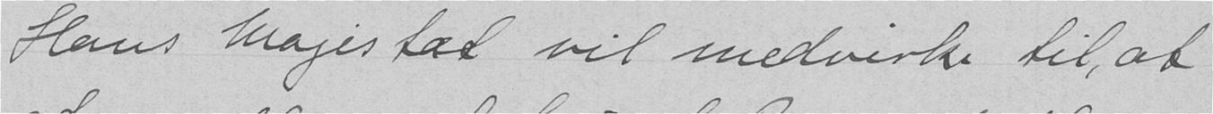Hans Majestæt vil medvirke til, at
